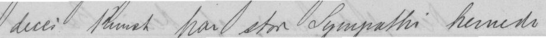Deres Kunst har stor Sympathi hernede
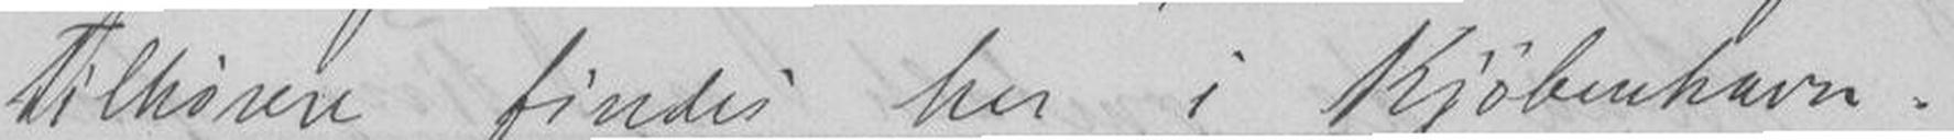Tilhørere findes her i Kjøbenhavn.
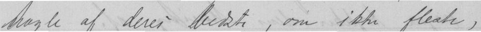nogle af deres bedste, om ikke fleste,
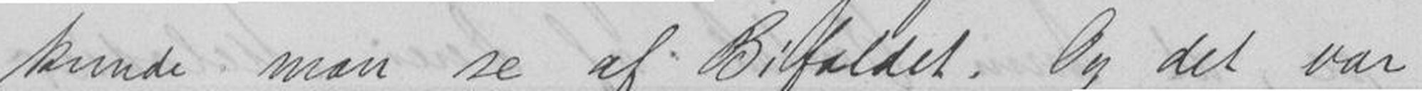kunde man se af Bifaldet. Og det var
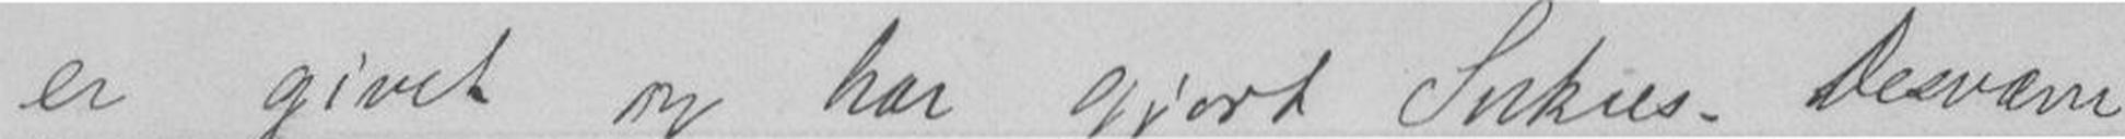er givet og har gjort Sukces Desværre
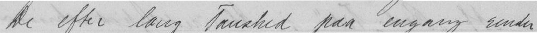De efter lang Taushed paa engang sender
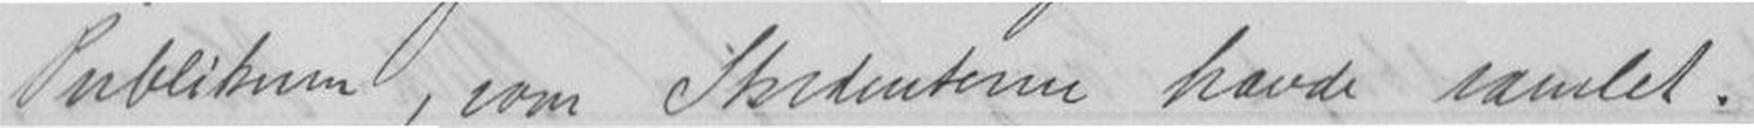Publikum, som Studentene havde samlet.
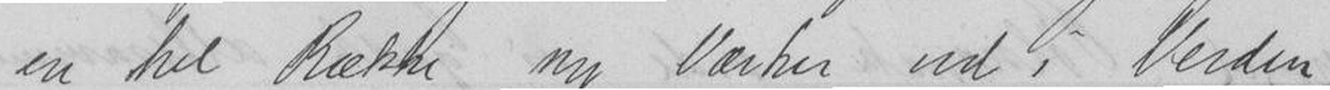en hel Række ny Værker ud i Verden
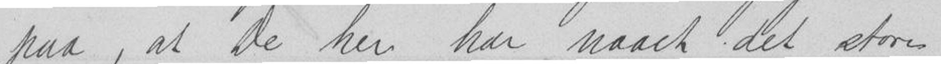paa, at De her har naaet det store
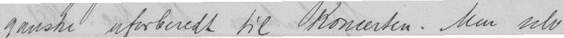ganske uforberedt til Koncerten kom selv
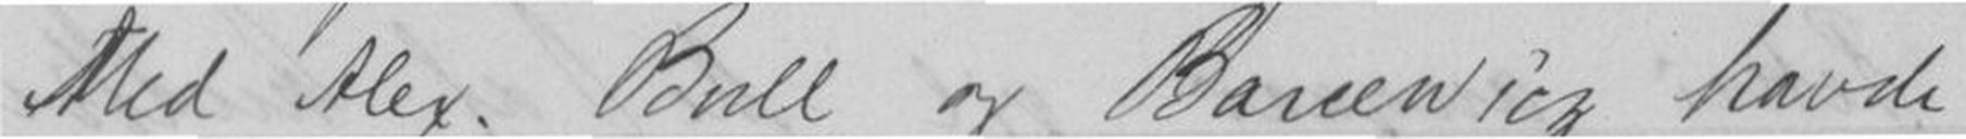Med Alex Bull og Barcewicz havde
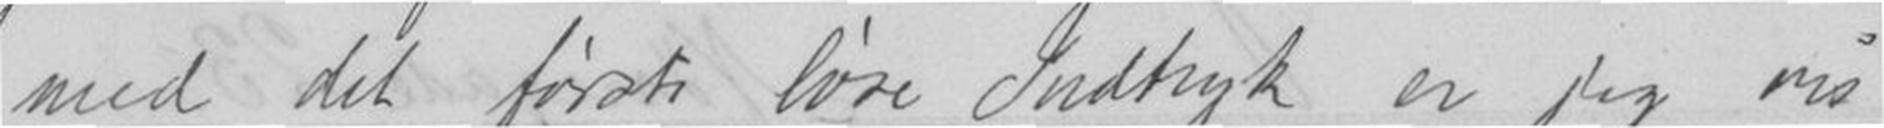med det første løse Indtryk er jeg vis
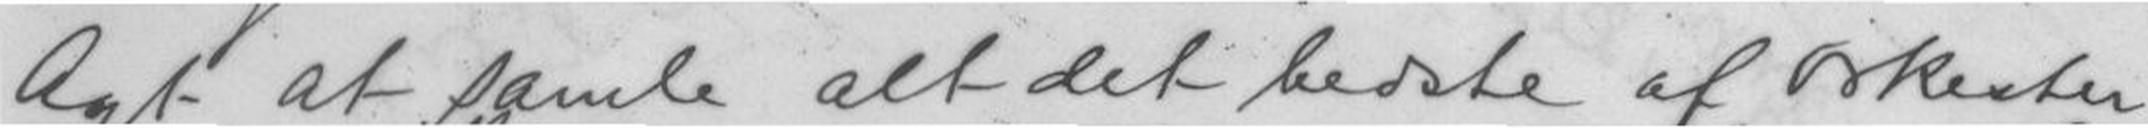Agt at samle alt det bedste af Orkester
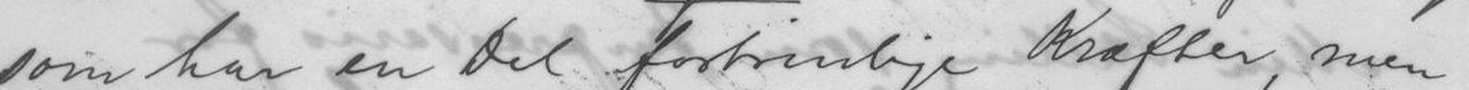som har en Del fortrinlige Kræfter, men
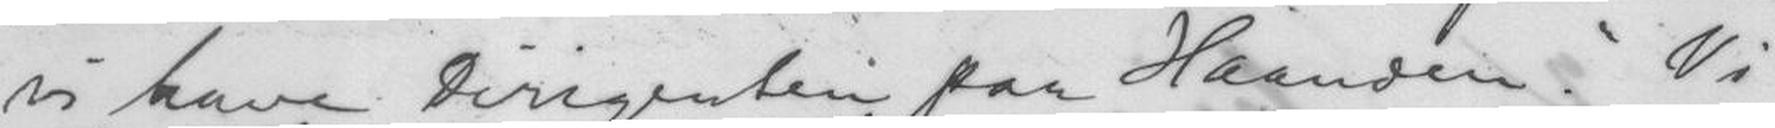vi have Dirigent paa Haanden. Vi
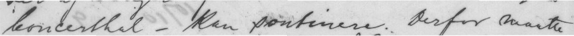Concerthal - kan sontinere. Derfor maatte
